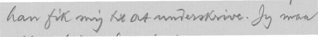han fik mig til at underskrive. Jeg maa
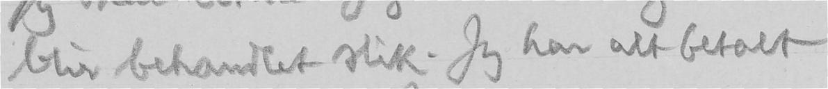blir behandlet slik. Jeg har alt betalt
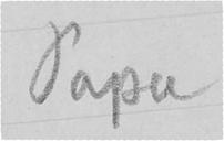Papa
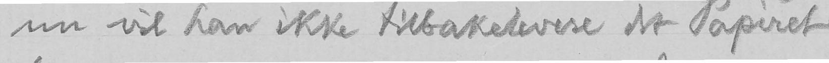nu vil han ikke tilbakelevere det Papiret
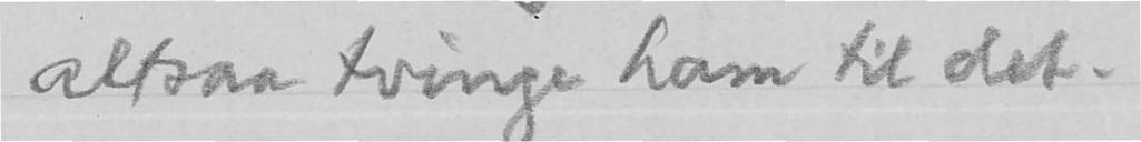altsaa tvinge ham til det.
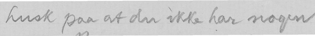husk paa at du ikke har nogen
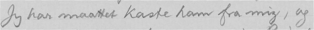Jeg har maattet kaste ham fra mig, og
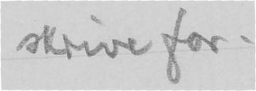skrive for.
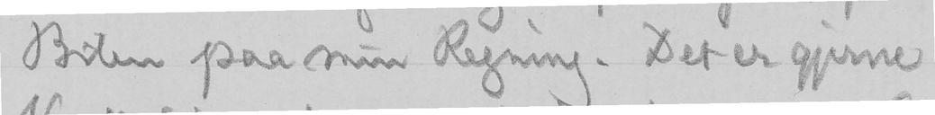Bilen paa min Regning. Det er gjerne
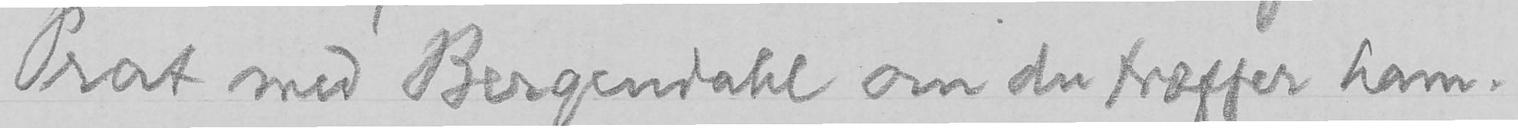Prat med Bergendahl om du træffer ham.
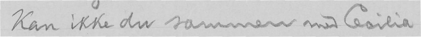Kan ikke du sammen med Cecilia
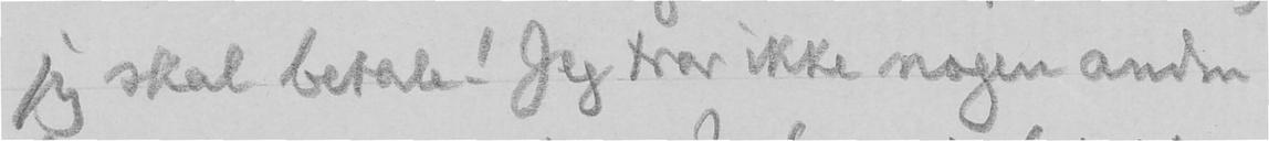jeg skal betale! Jeg tror ikke nogen anden
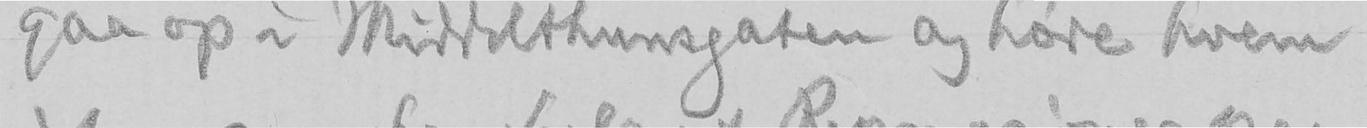gaa op i Middelthunsgaten og høre hvem
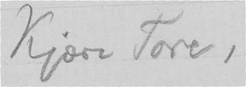Kjære Tore,
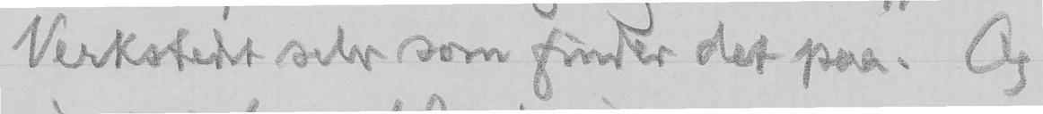Verkstedet selv som finder det paa. Og
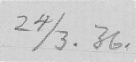24/3. 36.
